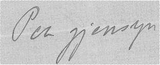Paa gjensyn
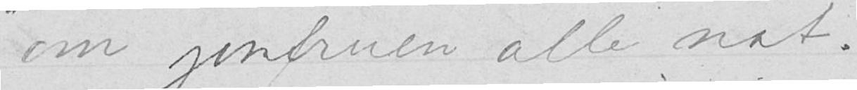"om jomfruen alle nat.
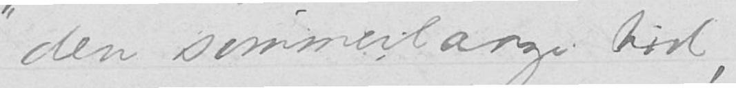"den sommerlange tid,
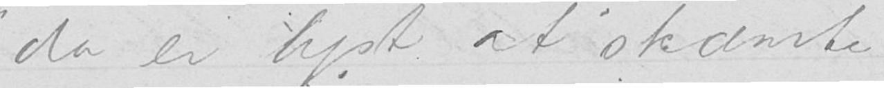"da er lyst at skæmte
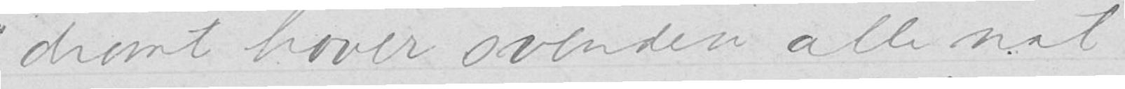"drømt haver svenden alle nat
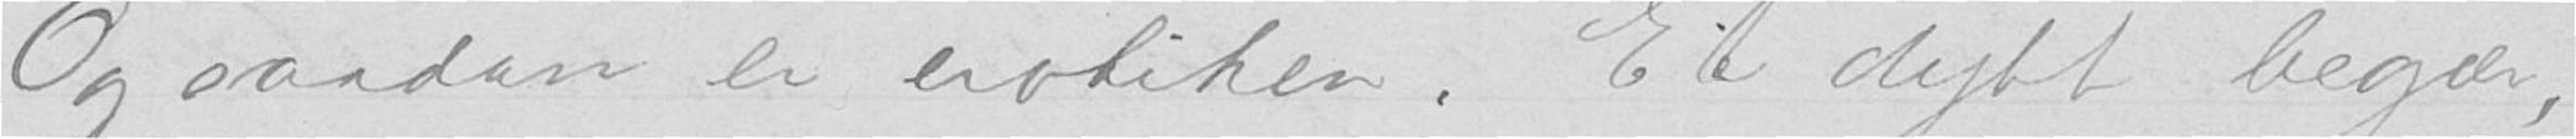Og saadan er erotiken. Et dybt begær,
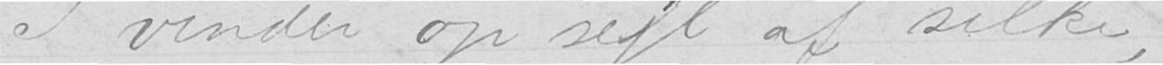"I vinder op sejl af silke,
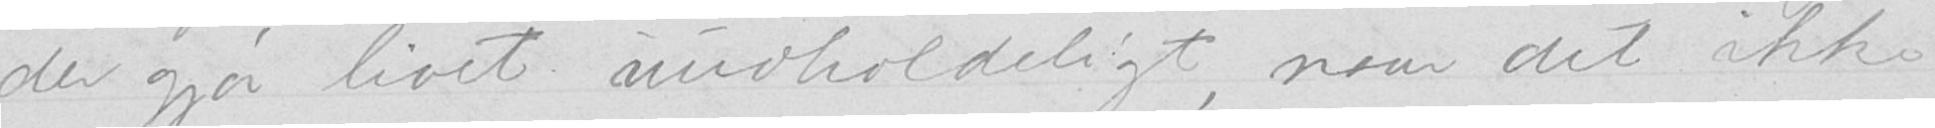der gjør livet uudholdeligt, naar det ikke
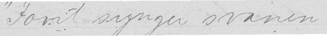"Fovit synger svanen
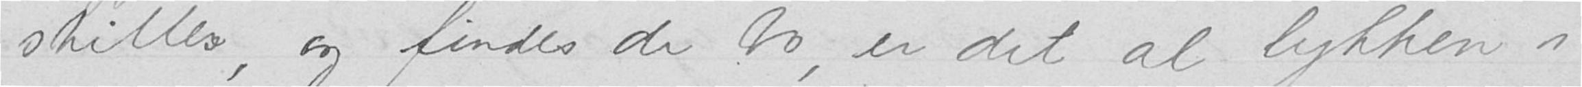stilles, og findes de to, er det al lykken i
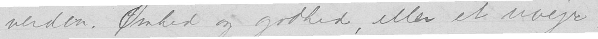verden. Ømhed og godhed, eller et uvejr,
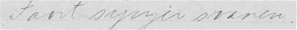"Fovit synger svanen!"
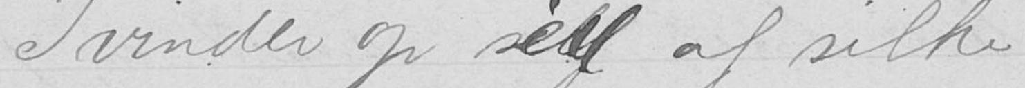"I vinder op sejl af silke,
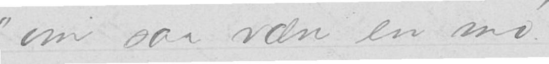"Om saa væn en mø.
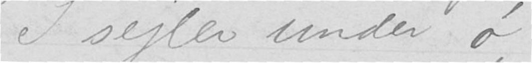"I sejler under ø,
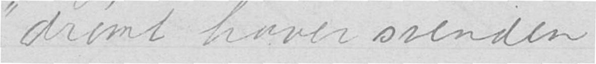"drømt haver svenden
